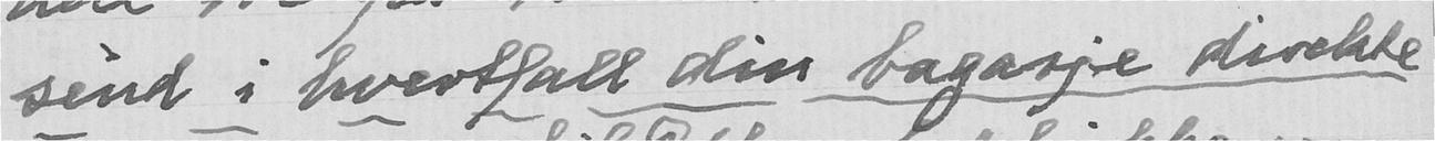send i hvertfall din bagasje direkte
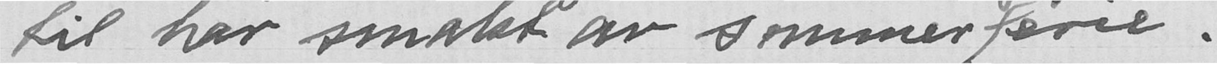til har smakt av sommerferie.
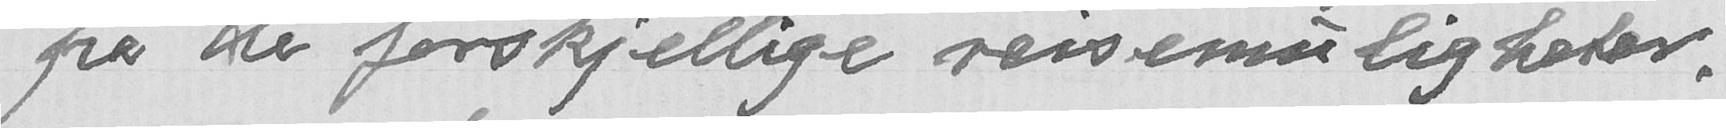på de forskjellige reisemuligheter,
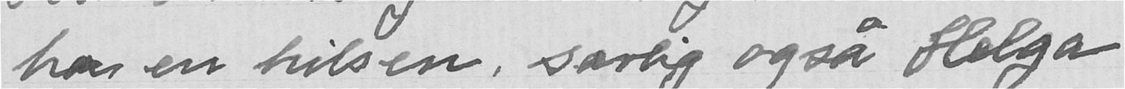ha en hilsen, særlig også Helga
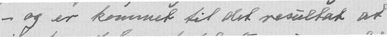- og er kommet til det resultat at
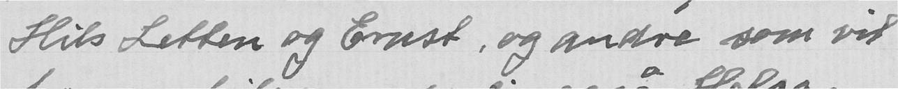Hils Letten og Ernst, og andre som vil
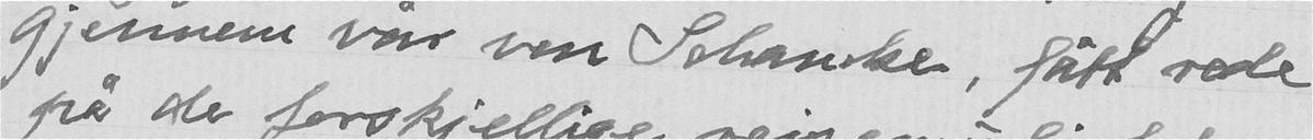gjennem vår ven Schanske, fått rede
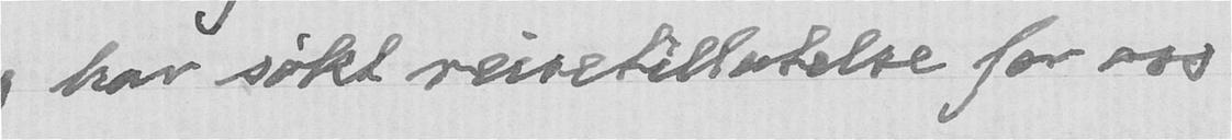Jeg har søkt reisetillatelse for oss
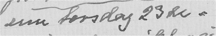enn torsdag 23de.
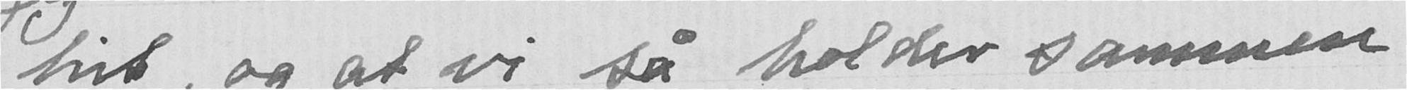hit, og at vi så holder sammen
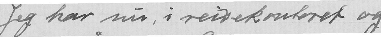Jeg har nu, i reisetontoret og
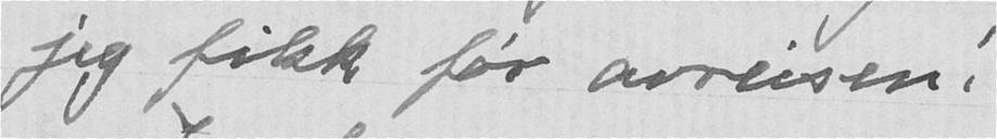jeg fikk før avreisen!
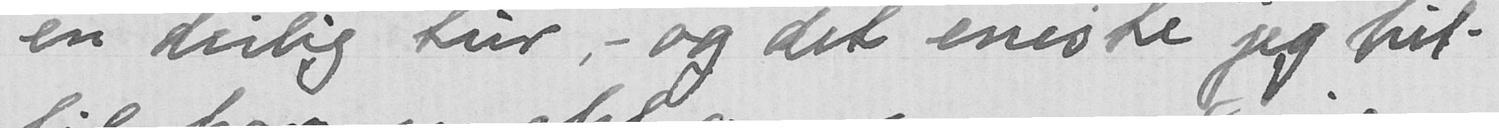en deilig tur,- og det eneste jeg hit-
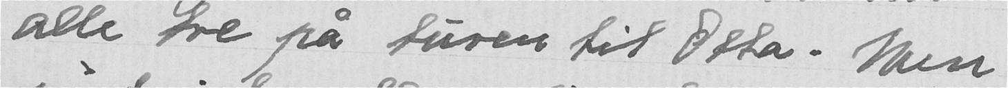alle tre på turen til Otta. Men
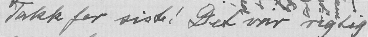Takk for sist! Det var rigtig
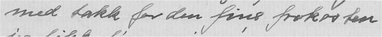med takk for den fine frokosten
